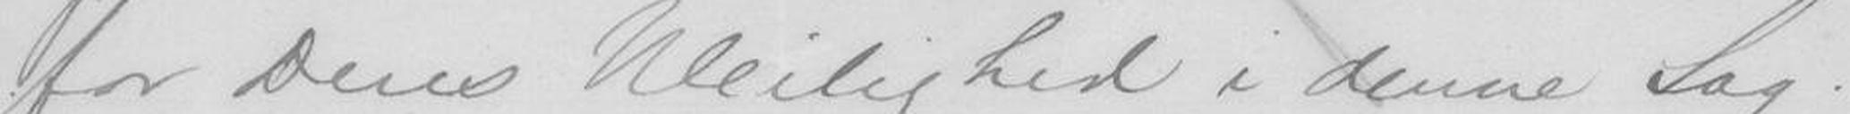for Deres Uleilighed i denne Sag
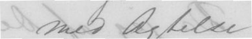med Agtelse
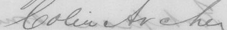Colin Archer
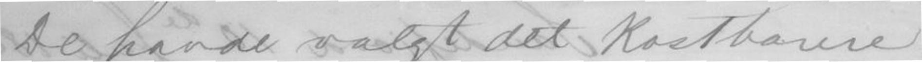De havde valgt det Kostbarere
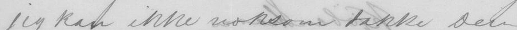jeg kan ikke noksom takke Dem
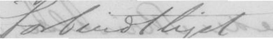Forbundtliget
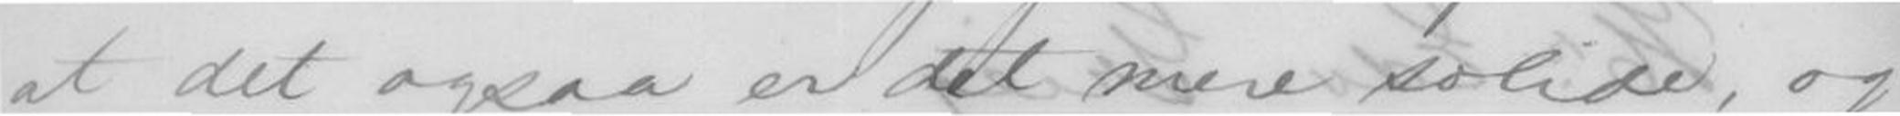at det ogsaa er det mere solide, og
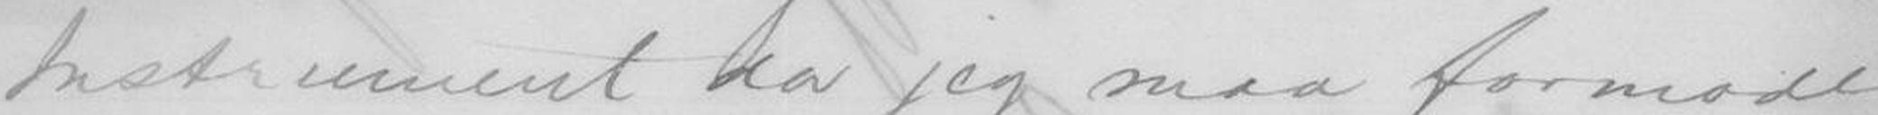Instrument da jeg maa formode
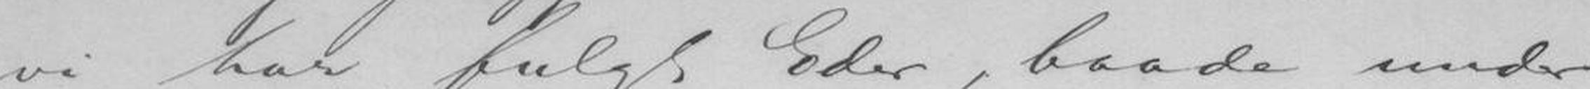vi har fulgt Eder, baade under
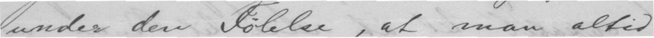under den Følelse, at man altid
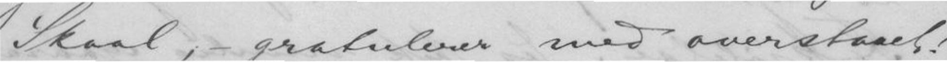Skaal, - gratulerer med overstaaet!
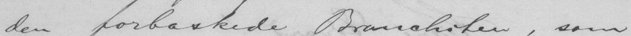den forbaskede Bronchiten, som
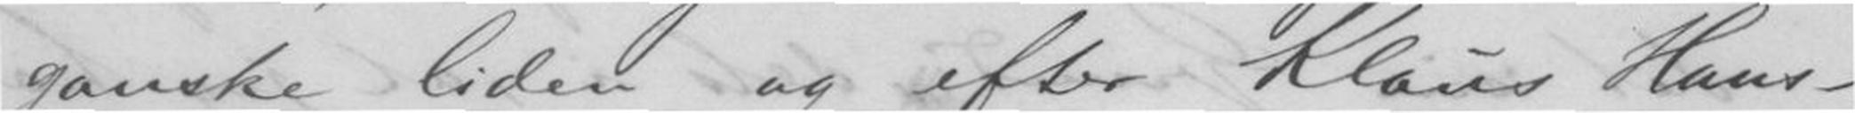ganske liden og efter Klaus Hans-
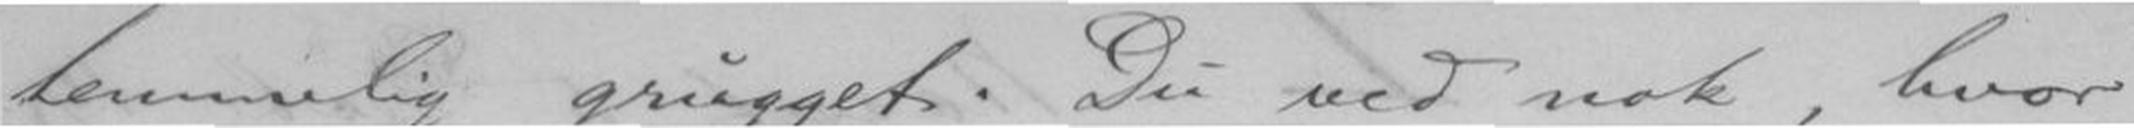temmelig grugget. Du ved nok, hvor
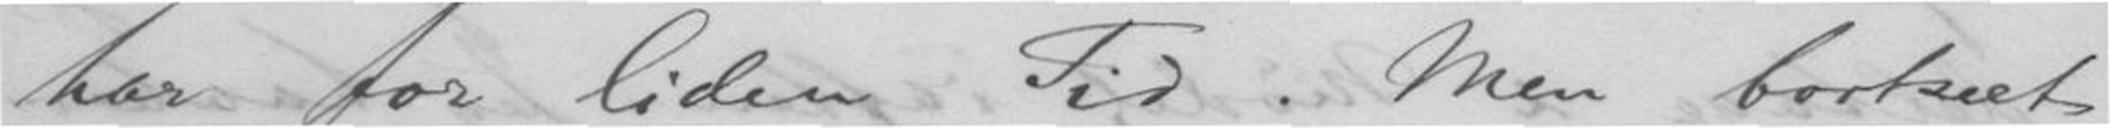har for liden Tid. Men bortseet
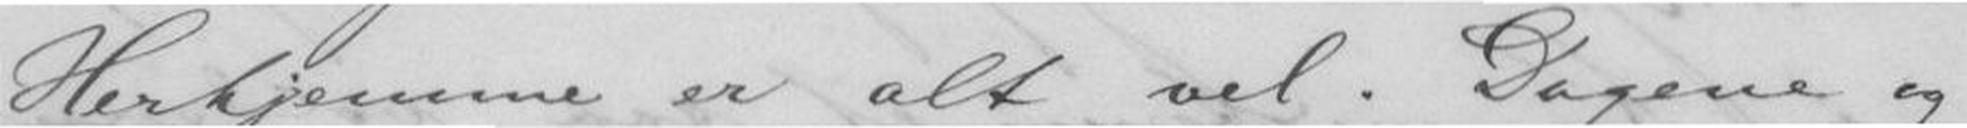- Herhjemme er alt vel. Dagene og
